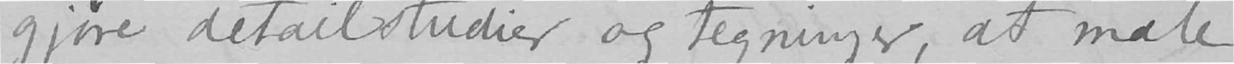gjøre detailstudier og tegninger, at male
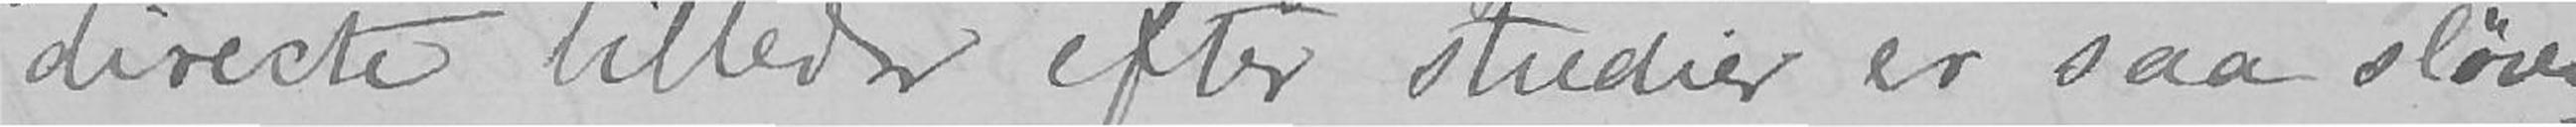directe billeder efter studier er saa sløven-
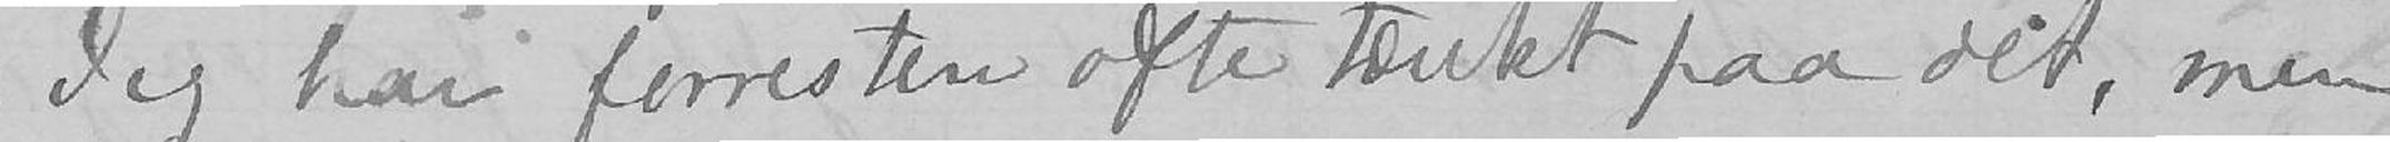Jeg har forresten ofte tænkt paa det, men
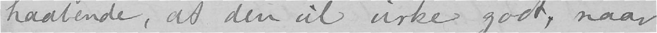haabende, at den vil virke godt, naar
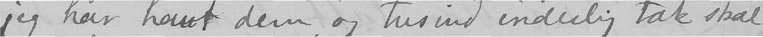jeg har havt dem, og tusind inderlig tak skal
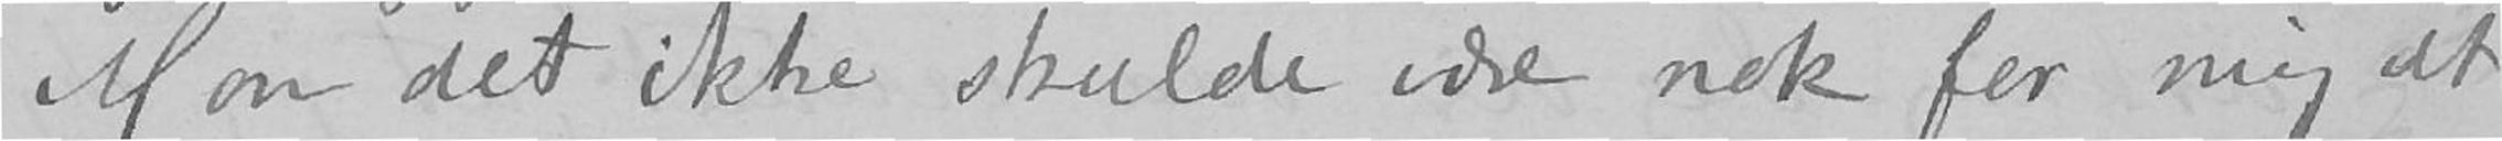Mon det ikke skulde være nok for mig at
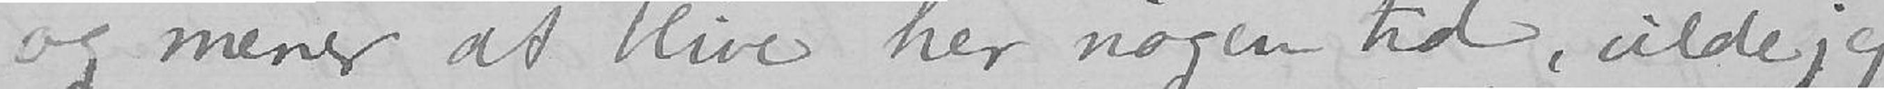og mener at blive her nogen tid, vilde jeg
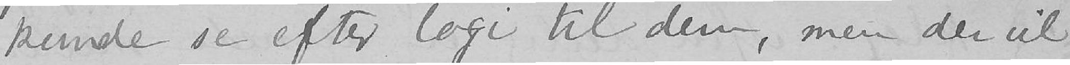kunde se efter logi til dem, men der vil
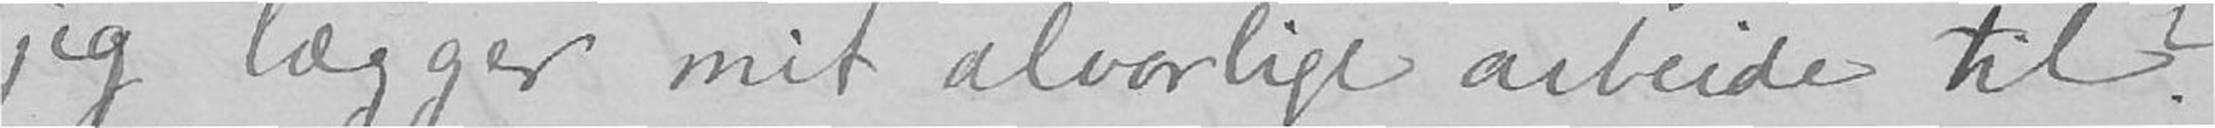jeg lægger mit alvorlige arbeide til.
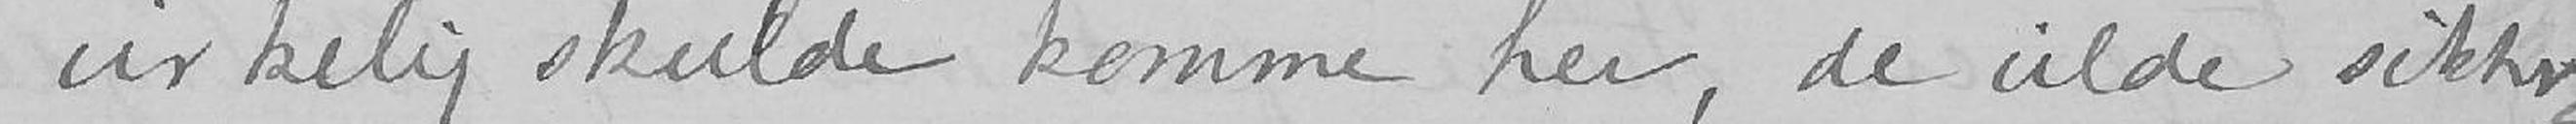virkelig skulde komme her, de vilde sikker-
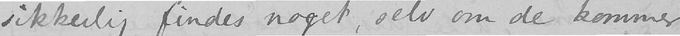sikkerlig findes noget, selv om de kommer
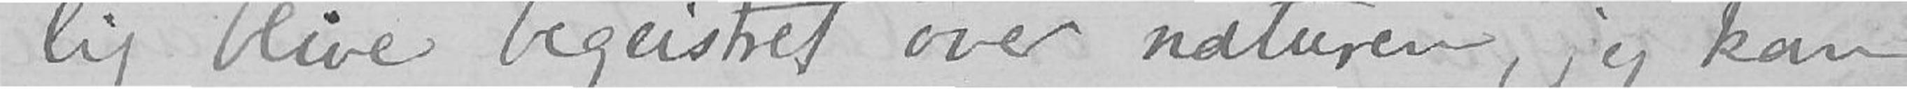lig blive begeistret over naturen, jeg kan
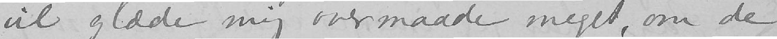vil glæde mig overmaade meget, om de
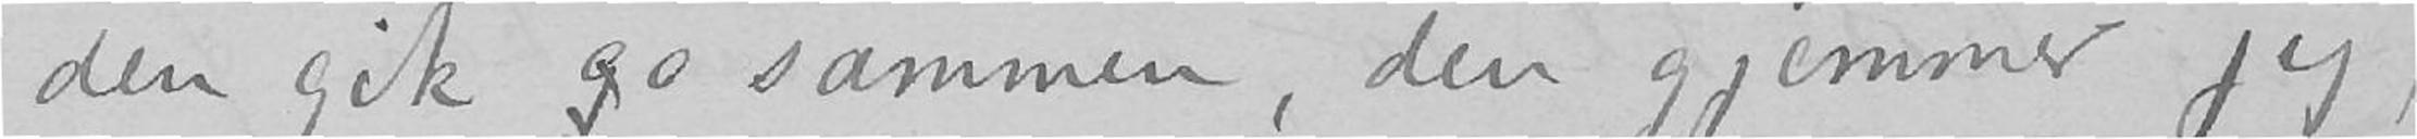den gik go sammen, den gjemmer jeg,
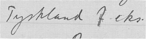Tyskland f.eks.
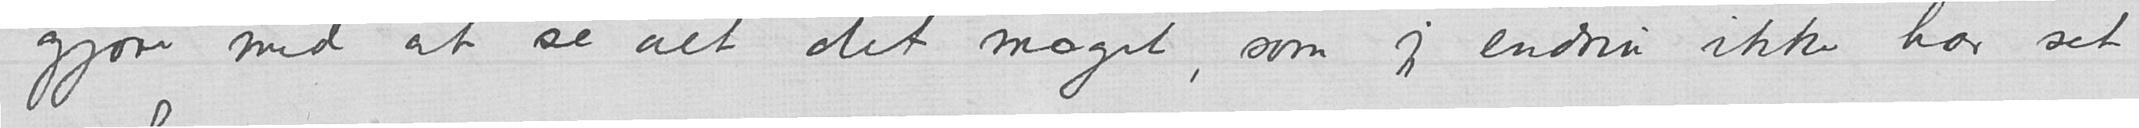gjøre med at se alt det moget, som jeg endnu ikke har set
